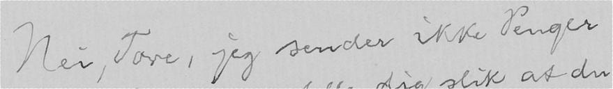Nei, Tore, jeg sender ikke Penger
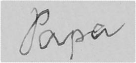Papa
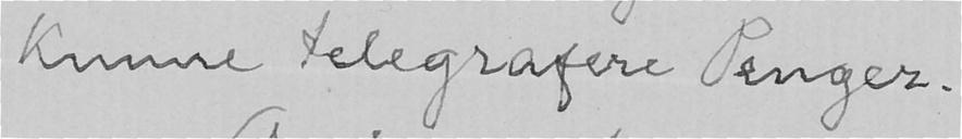kunne telegrafere Penger.
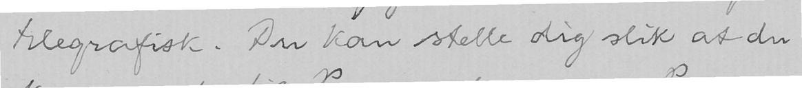telegrafisk. Du kan stelle dig slik at du
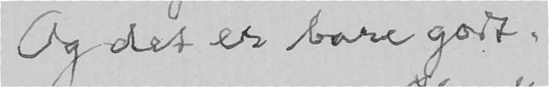Og det er bare godt.
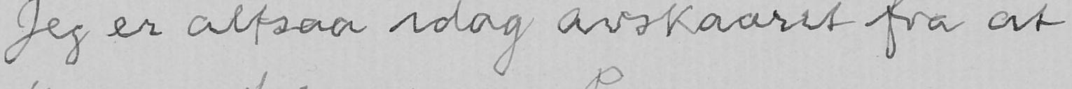Jeg er altsaa idag avskaaret fra at
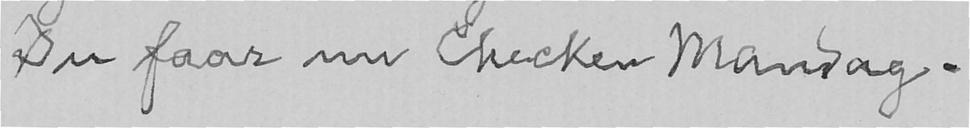Du faar nu Checken Mandag.
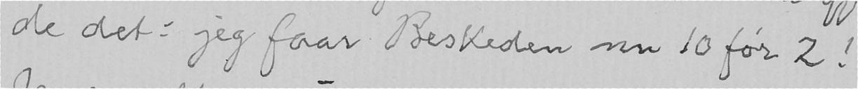de det: jeg faar Beskeden nu 10 før 2!
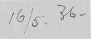16/5. 36.
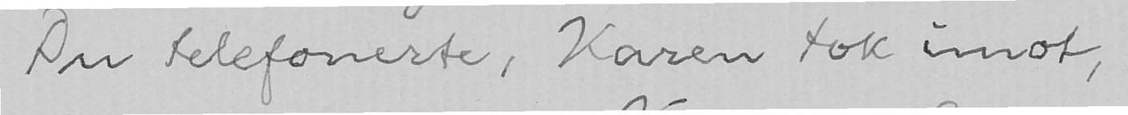Du telefonerte, Karen tok imot,
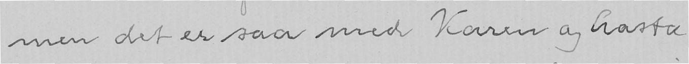men det er saa med Karen og Aasta
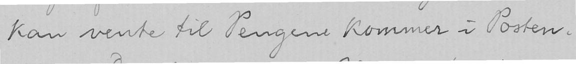kan vente til Pengene kommer i Posten.
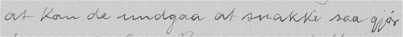at kan de undgaa at snakke saa gjør
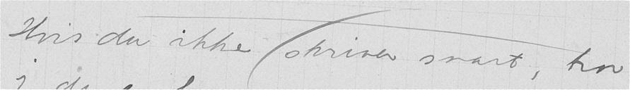Hvis du ikke skriver snart, tror
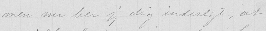men nu ber jeg dig inderligt, at
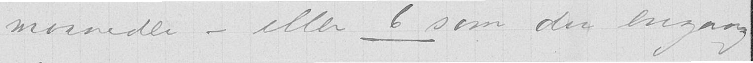maaneder — eller 6 som du engang
